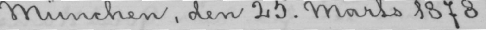München, den 25. Marts 1878.
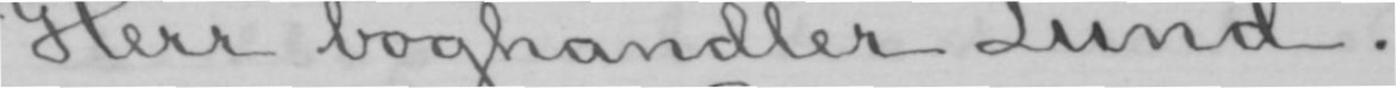Herr boghandler Lund.
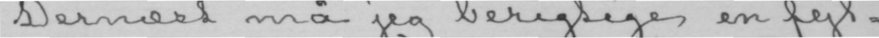Dernæst må jeg berigtige en fejl-
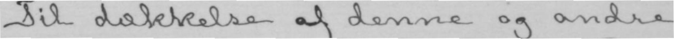Til dækkelse af denne og andre
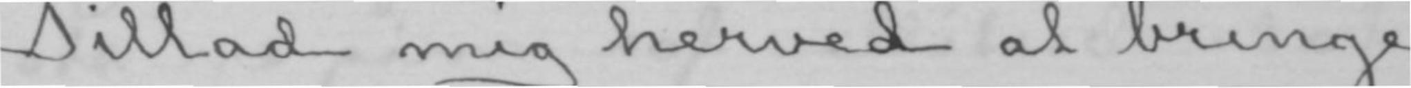Tillad mig herved at bringe
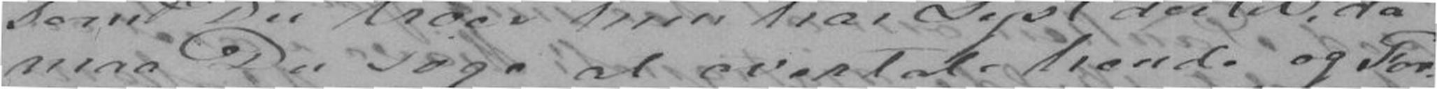maa Du søge at overtale hende og For-
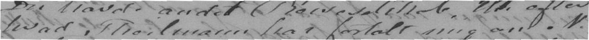hvad Theilmann har fortaldt mig om N.
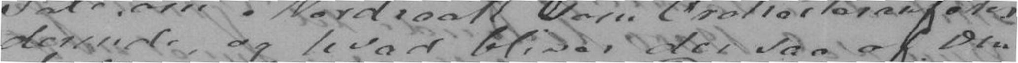dernede, og hvad bliver der saa af Din
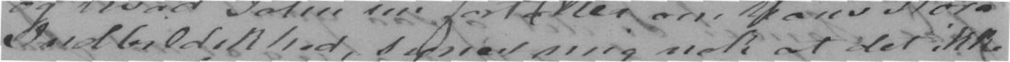Indbildskhed, synes mig nok at det ikke
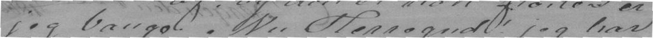jeg bange. Nu Herregud jeg har
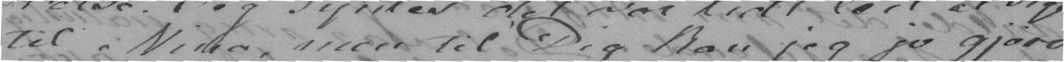til Nina, men til Dig kan jeg jo gjøre
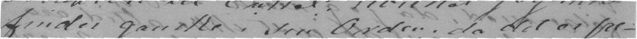finder ganske i sin Orden, da det er pre-
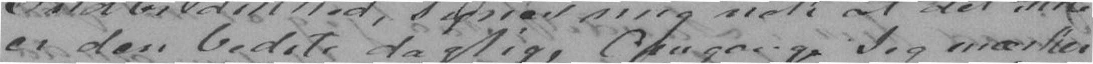er den bedste daglige Omgang. Jeg mærker
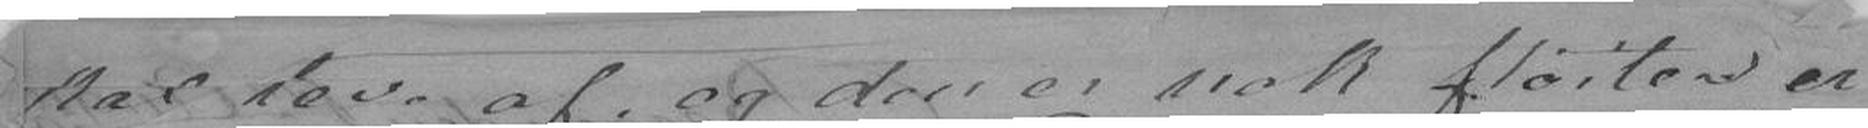skal leve af, og den er nok fløiten er
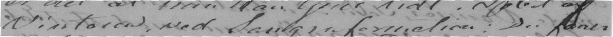Vinteren ved Sanginformation. Du faaer
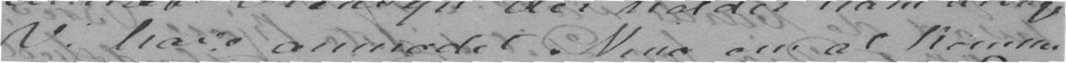Vi have anmodet Nina om at komme
